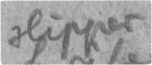slipper
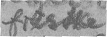friske
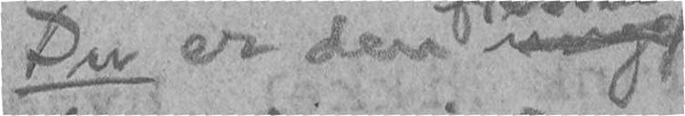Du er den unge
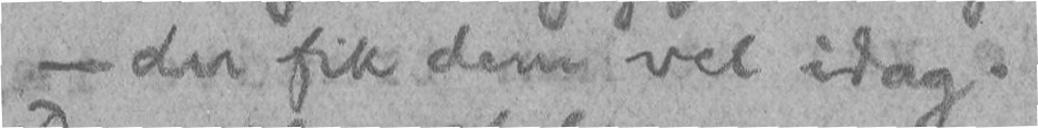- du fik dem vel idag.
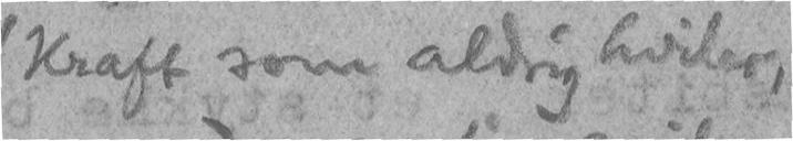Kraft som aldrig hviler,
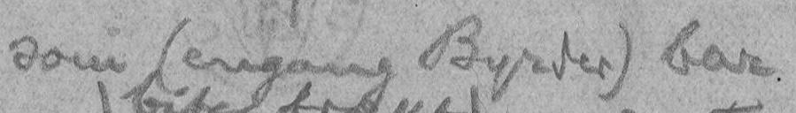som (engang Byrder) bare
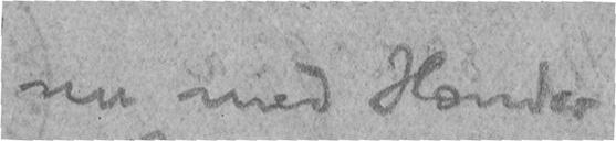nu med Hænder
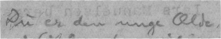Du er den unge Ælde,
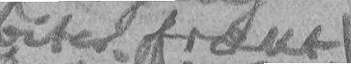biter frækt
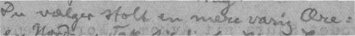Du vælger stolt en mere varig Ære:
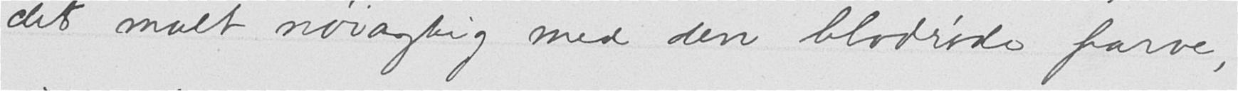det malt nøiagtig med den blodrøde farve,
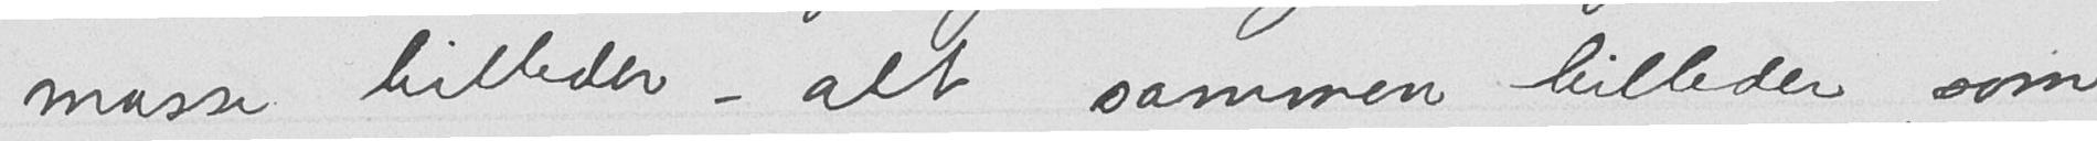masse billeder - alt sammen billeder, som
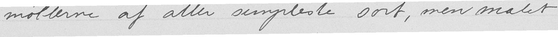møblerne af aller simpleste sort, men malet
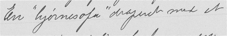En "hjørnesofa" draperet med et
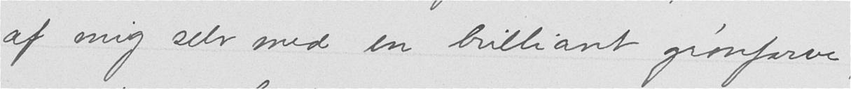af mig selv med en brilliant grønfarve,
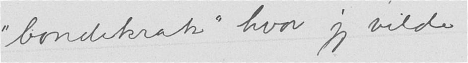"bondekrak" hvor jeg vilde
